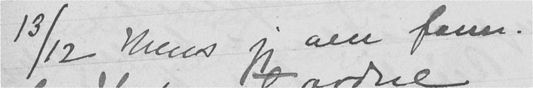13/12 Mens jeg om form.
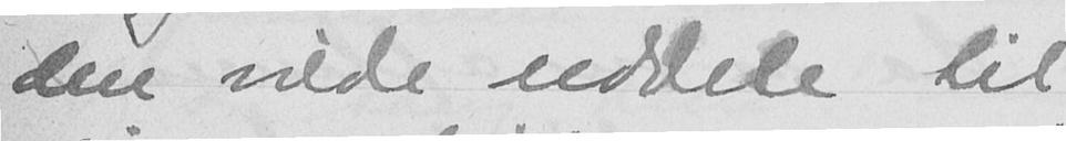den vilde uddele til
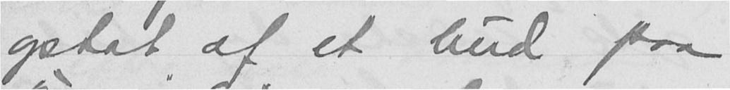optat af et bud paa
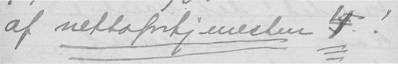af nettofortjenesten 4!
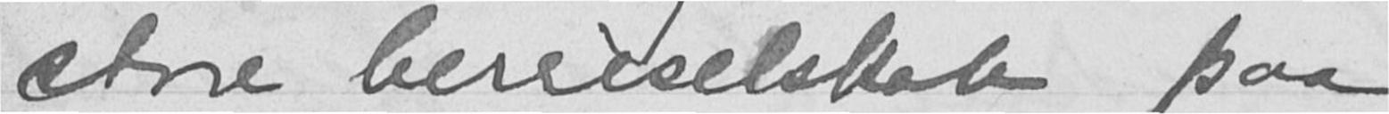store herreselskab paa
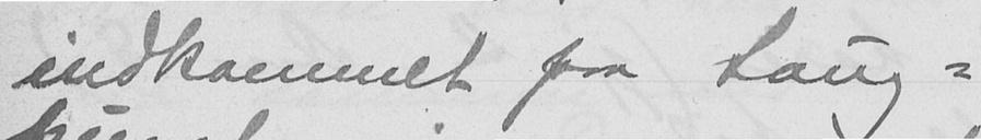indkommet paa Saug-
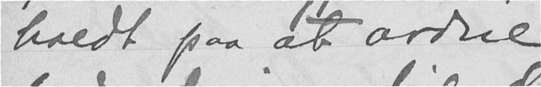holdt paa at ordne
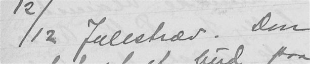12/12 Julestræv. Don
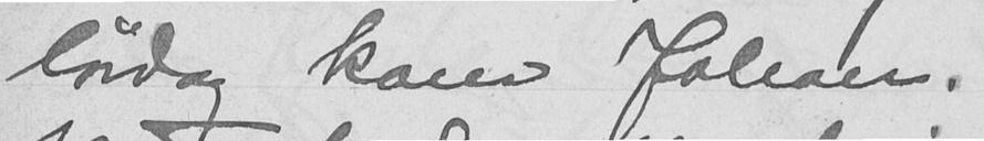lørdag kom Johan.
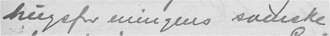brugsforeningens svenske
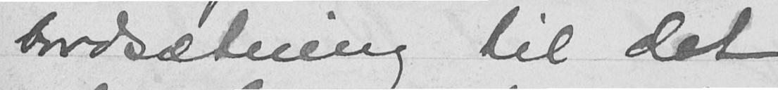bordsætning til det
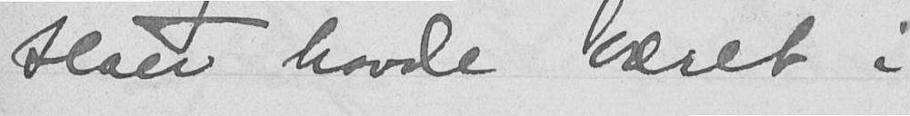Han havde været i
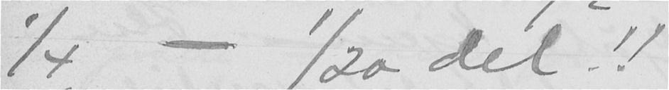¼ - 1/20 del!!
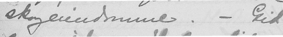skogeiendomme. - Gid
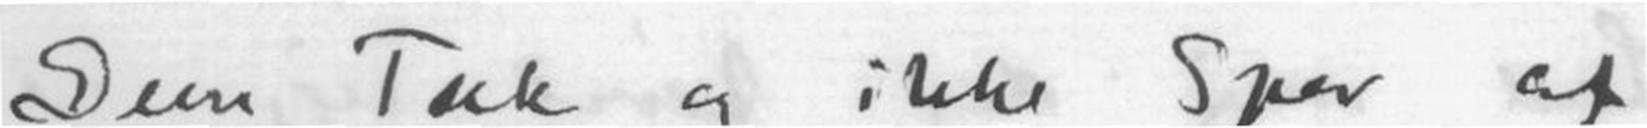Dem Tak og ikke spor af
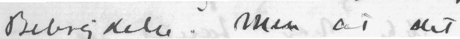Bebrejdelse. Men at det
